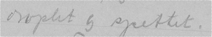droplet og spettet.
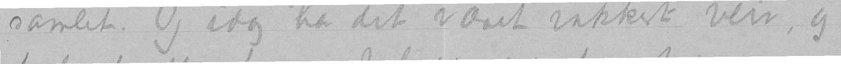samlet. Og idag har det været vakkert veir, og
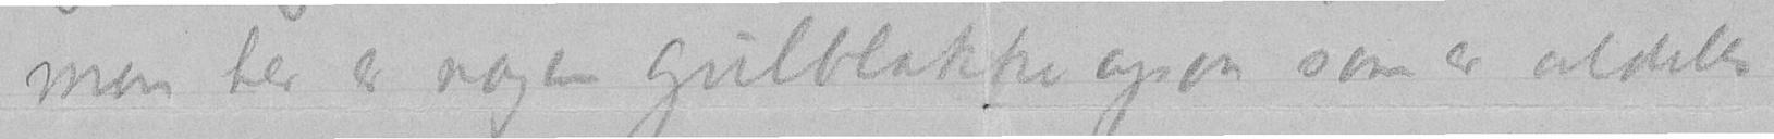men her er nogen gulblakke ogsaa som er aldeles
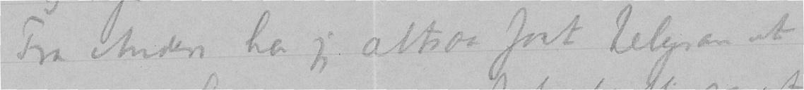Fra Anders har jeg altsaa faat telegram at
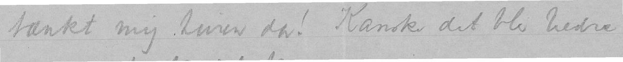tænkt mig turen da! Kanske det blir bedre
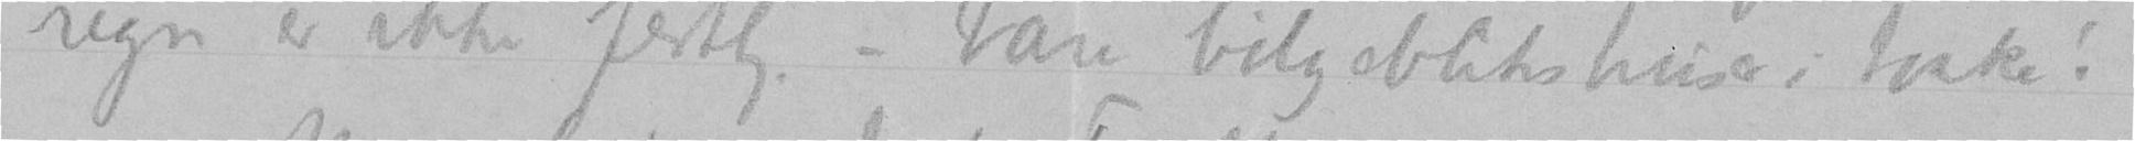regn er ikke festlig – bare bølgeblikshuser i taake!
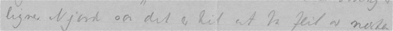ligner Njord saa det er til at ta feil av næsten,
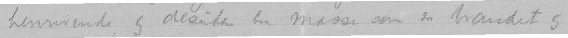henrivende, og desuten en masse som er brandet og
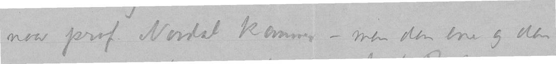naar prof. Nordal kommer – men den ene og den
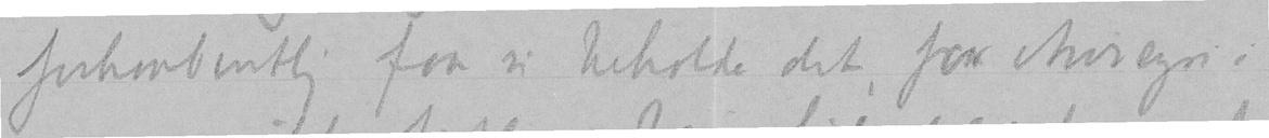forhaabentlig faar vi beholde det, for Akureyri i
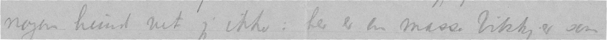nogen hund vet jeg ikke: her er en masse bikkjer som
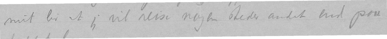mit liv at jeg vil reise nogen steder andet end paa
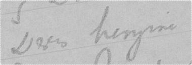Deres hengive
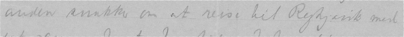anden snakker om at reise til Reykjavik med
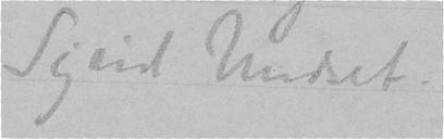Sigrid Undset.
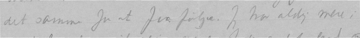det samme for at faa følge. Jeg tror aldrig mere i
